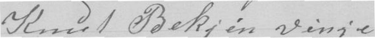Knut Bekjin Vinje
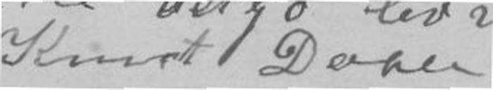Knut Dahle
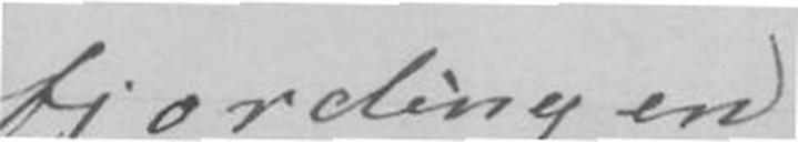fjordingen
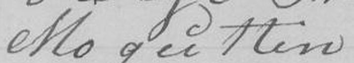Mo gutten
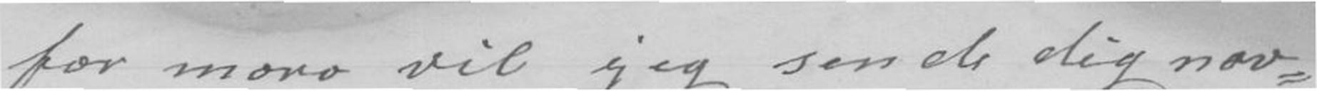for moro vil jeg sende dig nav-
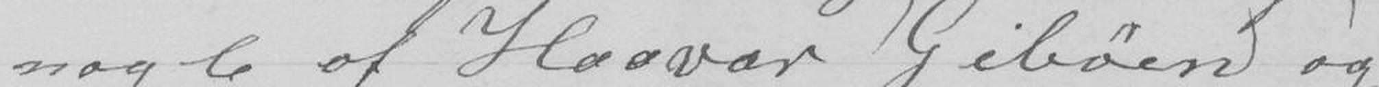nogle af Haavar Gibøen og
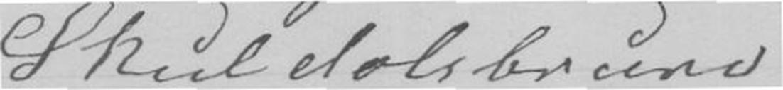Skuldalsbrune
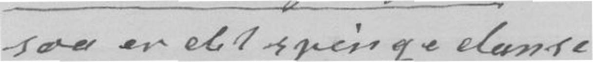saa er det spinge danse
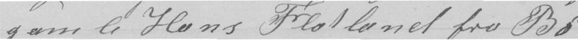gamle Hans Flatland fra Bø
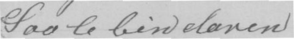Saalebindaren
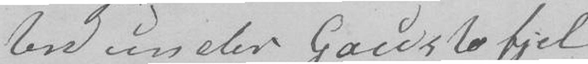ten under Gaustafjel
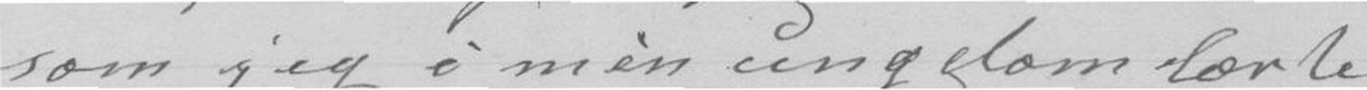som jeg i min ungdom lærte
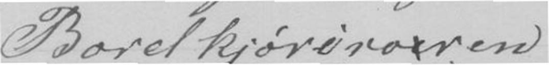Bordkjøriraren
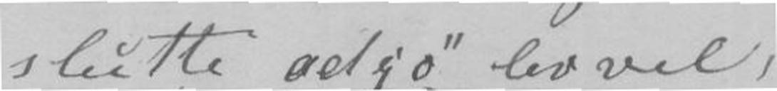slutte adjø levvel,
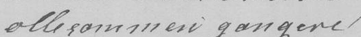allesammen gangere
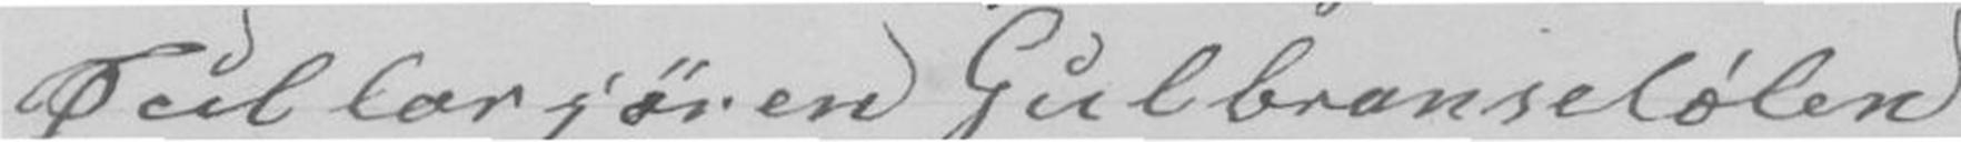Jullarjøren Gulbransdølen
